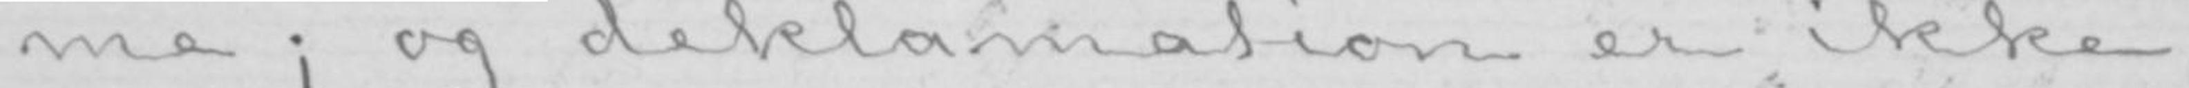me; og deklamation er ikke
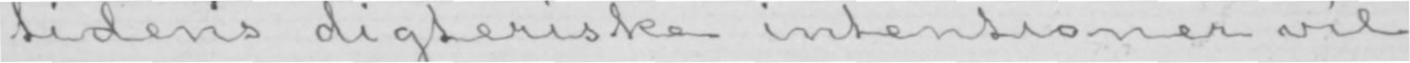tidens digteriske intentioner vil
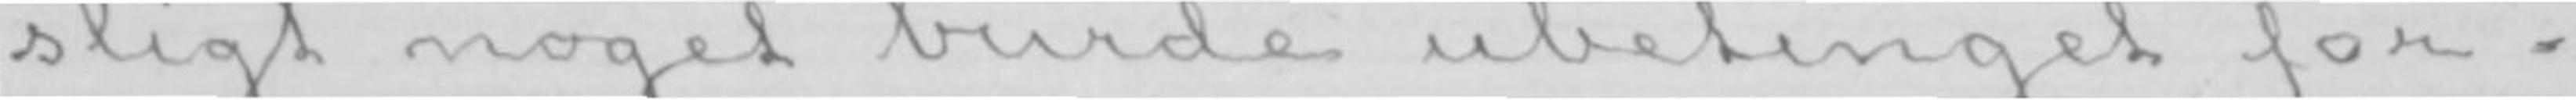sligt noget burde ubetinget for-
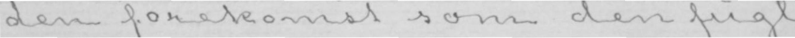den forekomst som den fugl
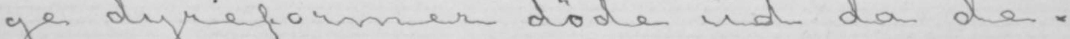ge dyreformer døde ud da de-
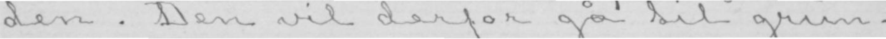den. Den vil derfor gå til grun-
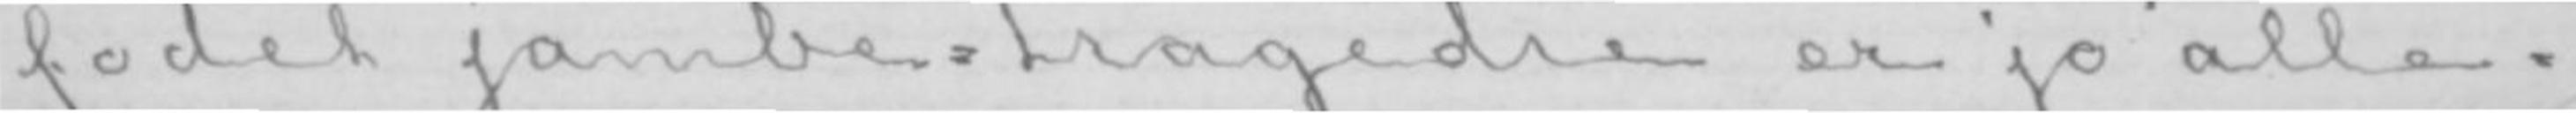fodet jambe-tragedie er jo alle-
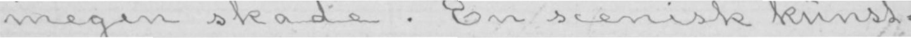megen skade. En scenisk kunst-
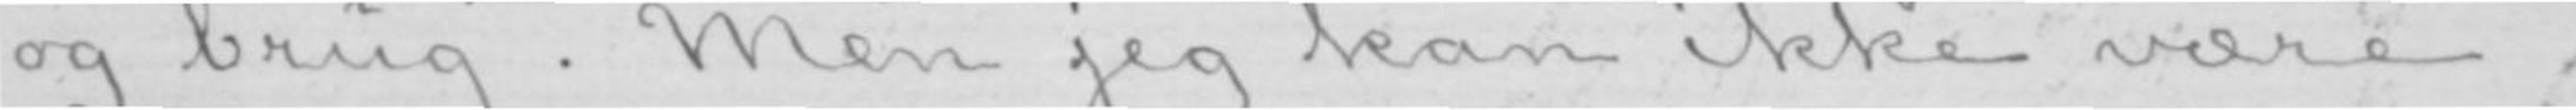og brug. Men jeg kan ikke være
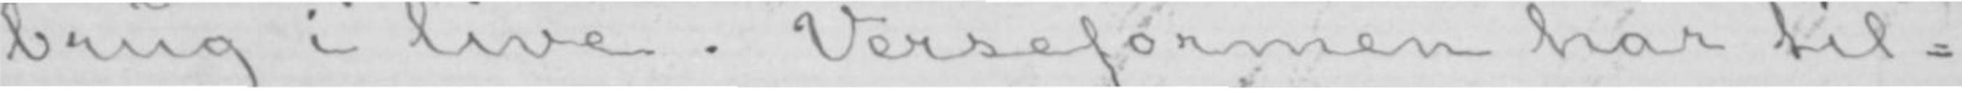brug i live. Verseformen har til-
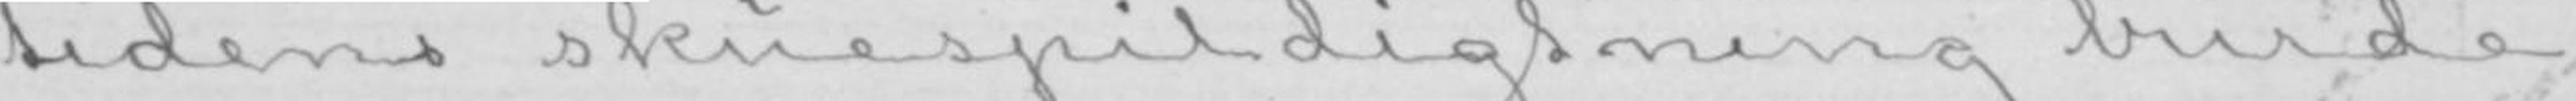tidens skuespildigtning burde
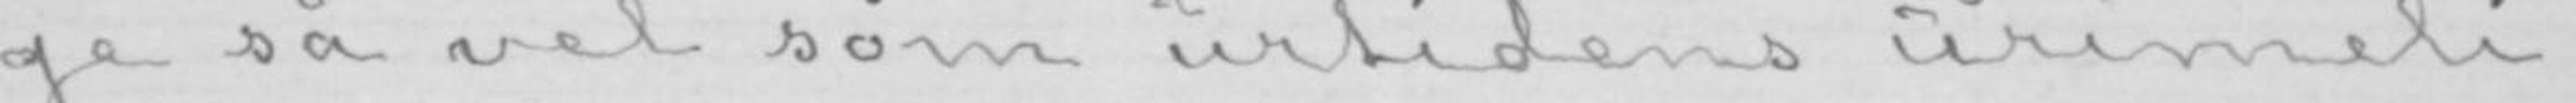ge så vel som urtidens urimeli-
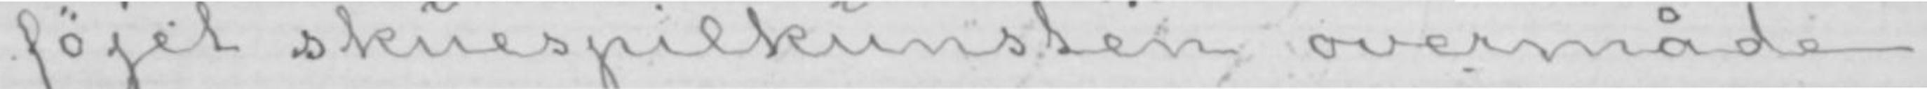føjet skuespilkunsten overmåde
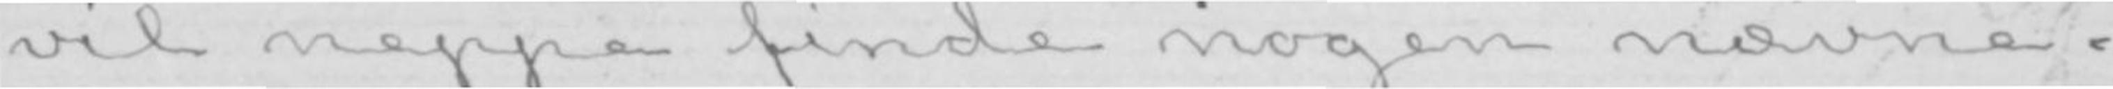vil neppe finde nogen nævne-
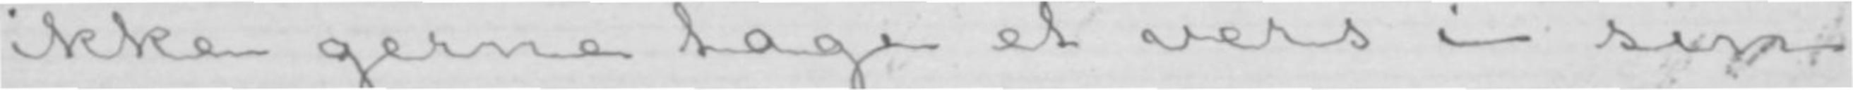ikke gerne tage et vers i sin
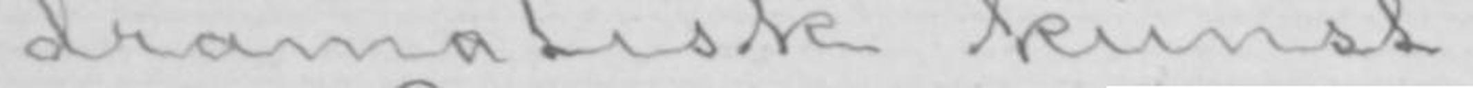dramatisk kunst.
